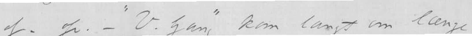osv.osv. - "V. Gang" kom langt om længe
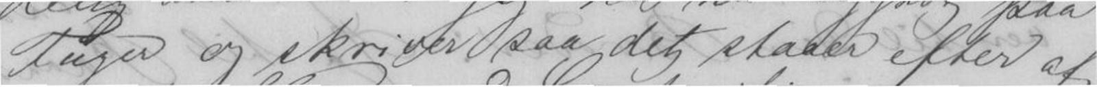Fuger og skriver saa det staar efter af
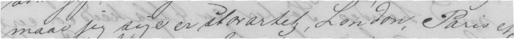maa jeg sige er storartet, London, Paris etc.
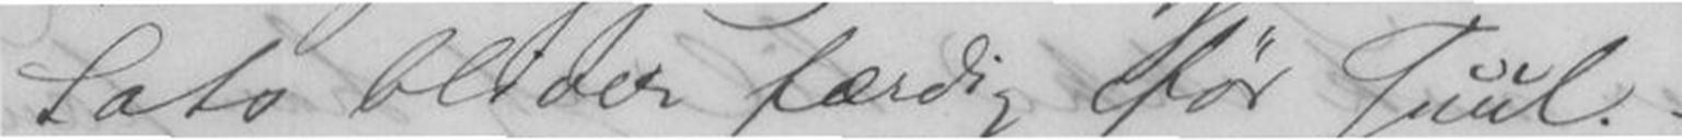Sats bliver færdig før Jul.
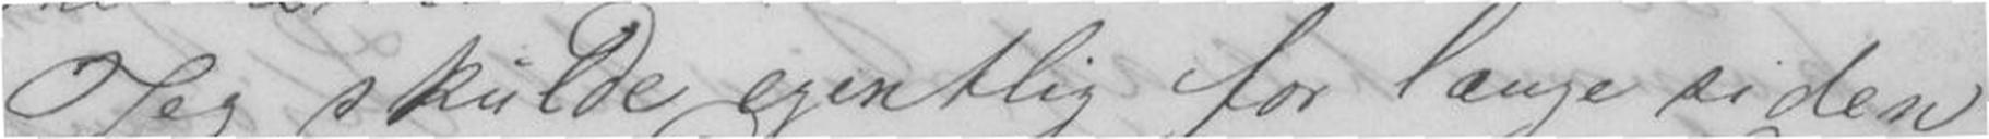Jeg skulde egentlig for længe siden
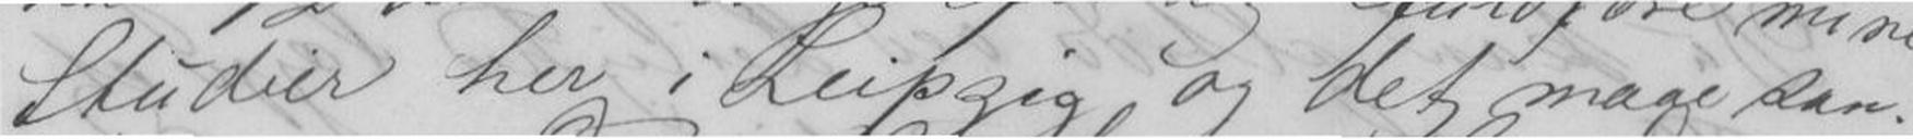Studier her i Leipzig og det maa san-
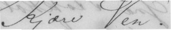Kjære Ven!
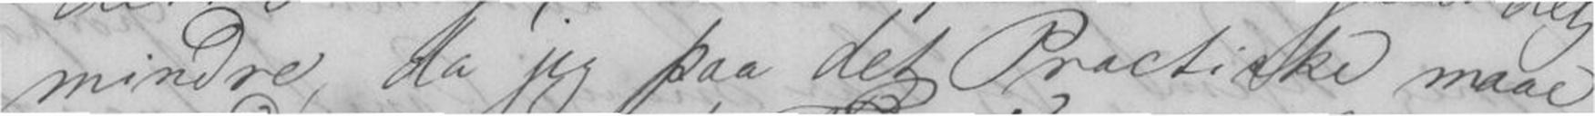mindre, da jeg paa det Practiske maae
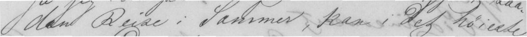dan Reise i Sommer, kan i Det høieste
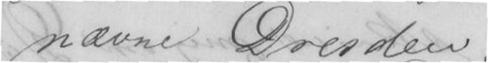nævne Dresden.
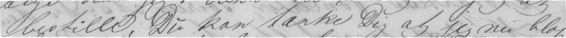bestille, Du kan tænke Dig at jeg nu blot
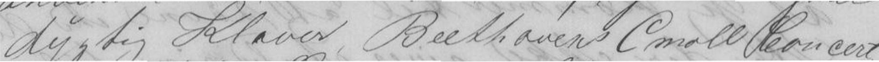dygtig Klaver Beethovens C moll Concert
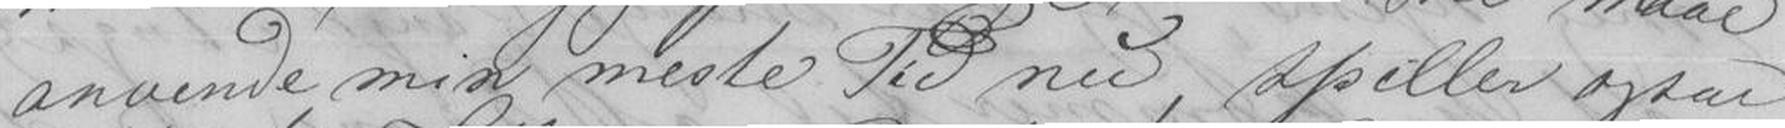anvende min meste Tid nu, spiller ogsaa
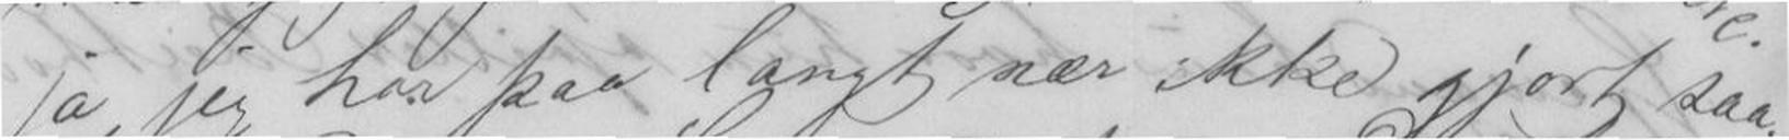ja jeg har paa langt nær ikke gjort saa-
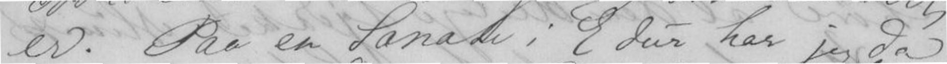er. Paa en Sonate i E dur har jeg da
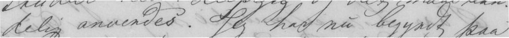delig anvendes. Jeg har nu begyndt paa
